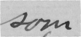som
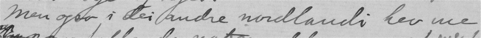Men ogso i dei andre nordlandi hev me
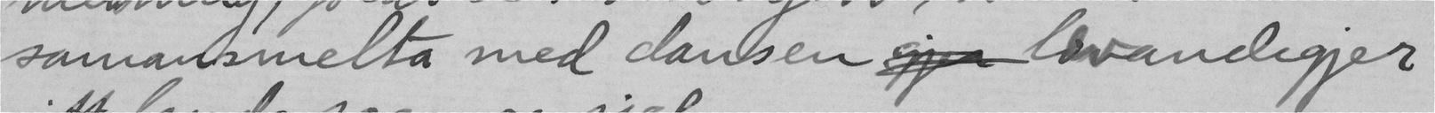samansmelta med dansen gjer levandegjer
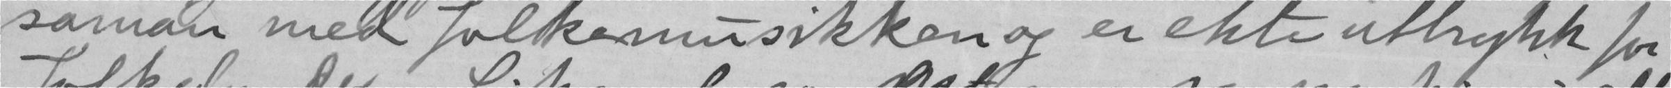saman med folkemusikken og er ekte uttrykk for
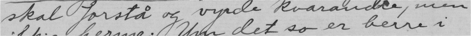skal forstå og vyrde kvarandre, men
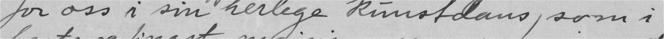for oss i sin herlege kunstdans, som i
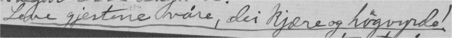Leve gjestene våre, dei kjære og høgvyrde!
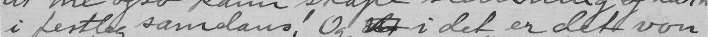i festleg samdans! Og det i det er det von
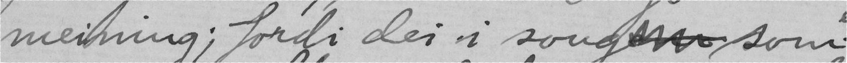meining; fordi dei i songer som
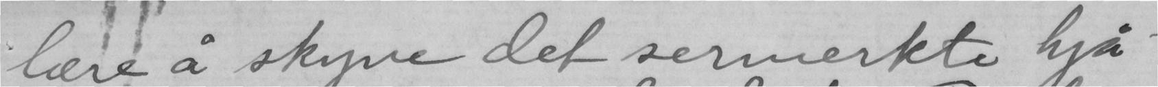lære å skyve det sermerkte hjå
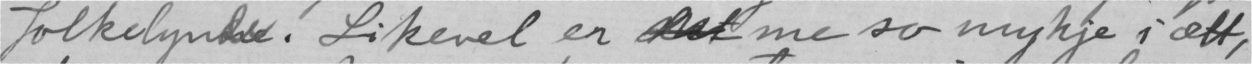folkelynne. Likevel er det me so mykje i ætt,
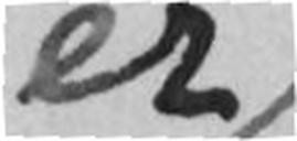er
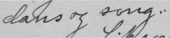dans og song.
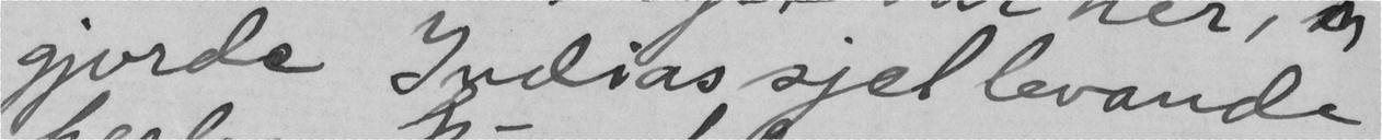gjorde Indias sjel levande
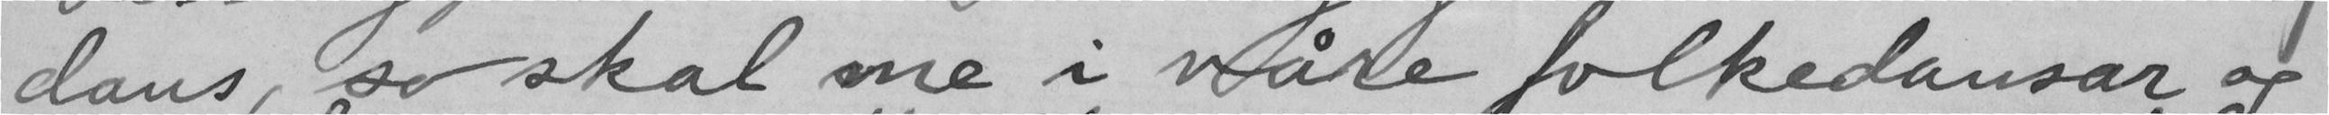dans, so skal me i våre folkedansar og
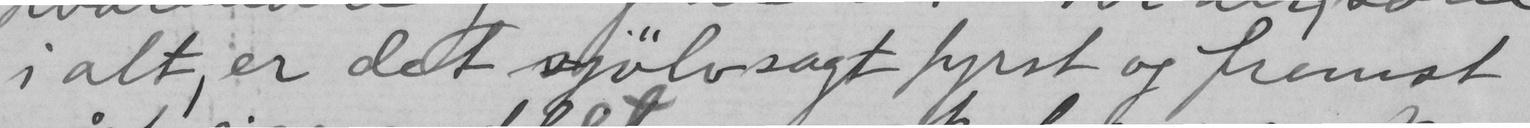i alt, er det sjølvsagt fyrst og fremst
